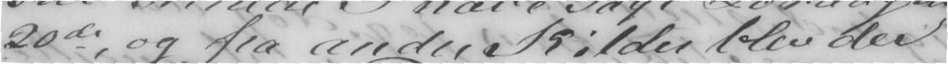20de, og fra andre Kilder blev der
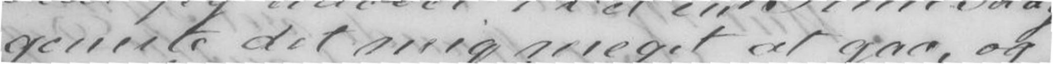generte det mig meget at gaa, og
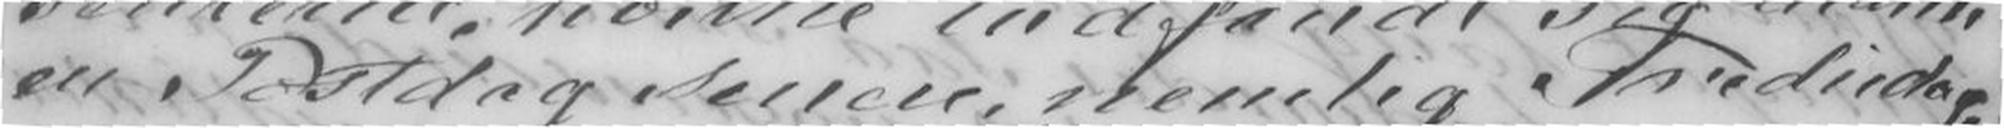en Postdag senere, nemlig Trediedag,
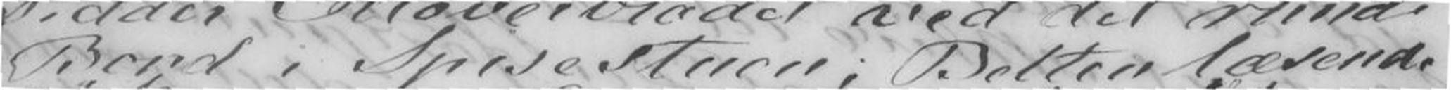Bord i Spisestuen; Betten læsende
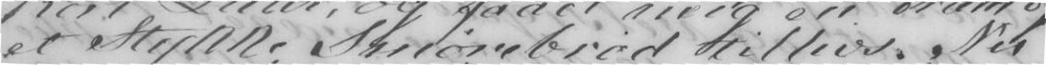et Stykke Smørrebrød tillivs. Nu
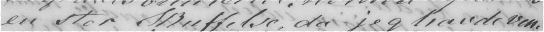en stor skuffelsem da jeg havde ven-
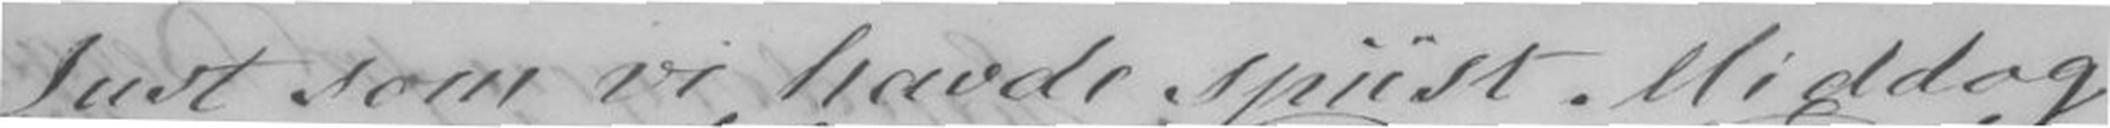Just som vi havde spiist Middag
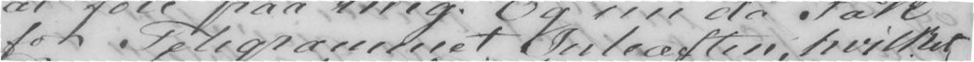for Telegrammet Juleaften, hvilket
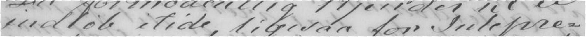indløb itide, ligesaa for Julepre-
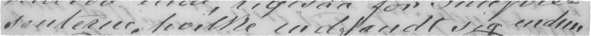senterne, hvilket indfant sig endnu
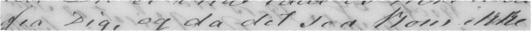fra Dig, og da det saa kom ikke
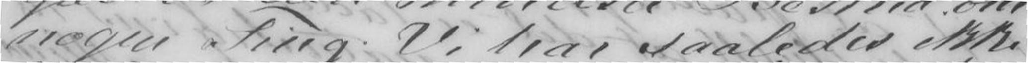nogen Ting. Vi har saaledes ikke
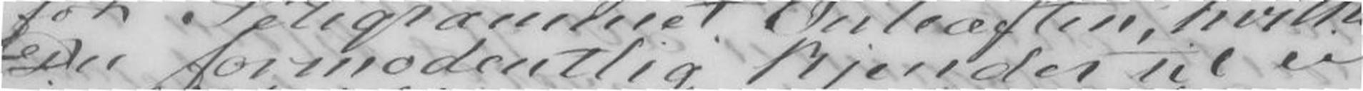Du formodentlig kjender til ei
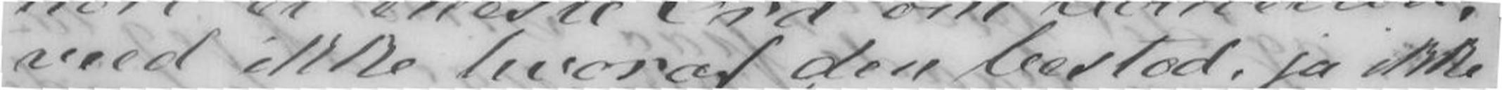veed ikke hvoraf den bedste, ja ikke
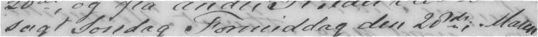sagt Søndag Formiddag de 28de; Maren
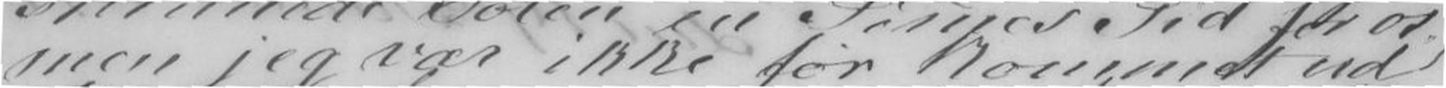men jeg var ikke før kommet ud
